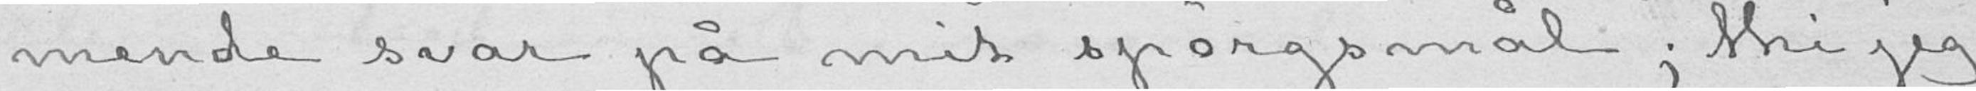mende svar på mit spørgsmål; thi jeg
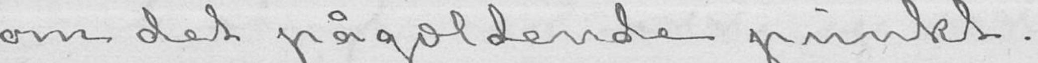om det pågældende punkt.
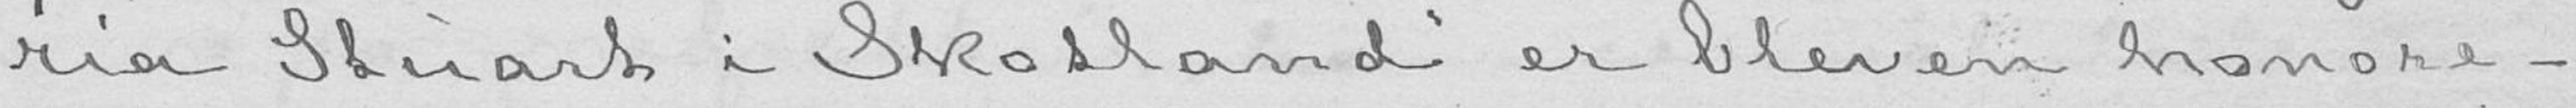ria Stuart i Skotland» er bleven honore-
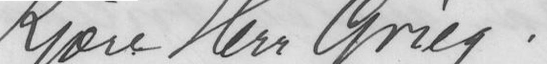Kjære Herr Grieg!
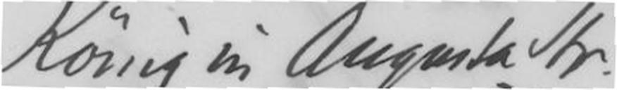Königin Augusta Str.
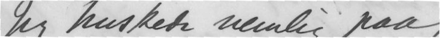Jeg husket nemlig paa,
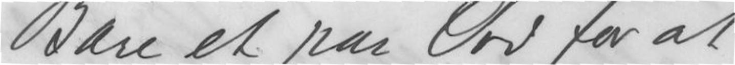Bare et par Ord for at
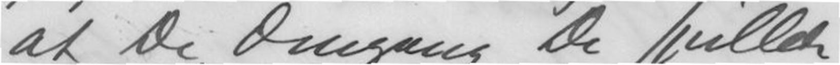at De, dengang De spillede
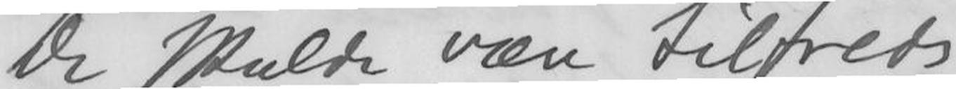De skulde være tilfreds
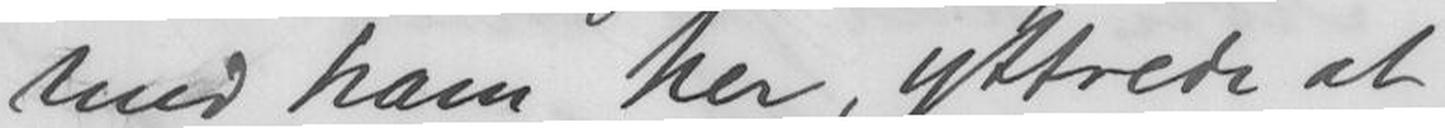med ham her, yttrede at
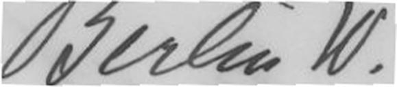Berlin W.
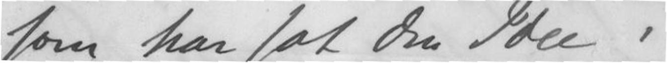som har sat den Idee i
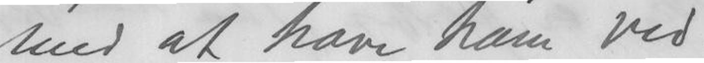med at have ham ved
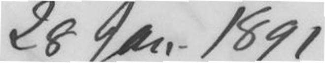28 Jan. 1891
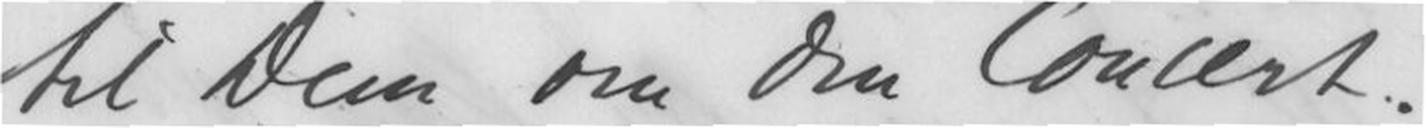til Dem om den Concert.
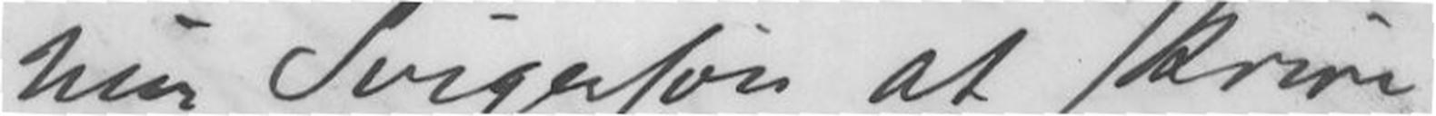min Svigerfar at skrive
